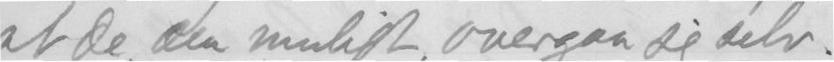at de, om muligt, overgaa sig selv.
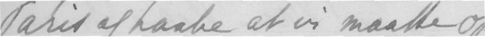Paris og haabe at vi maatte op-
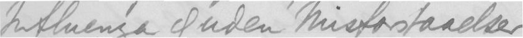Influenza og uden Misforstaaelser
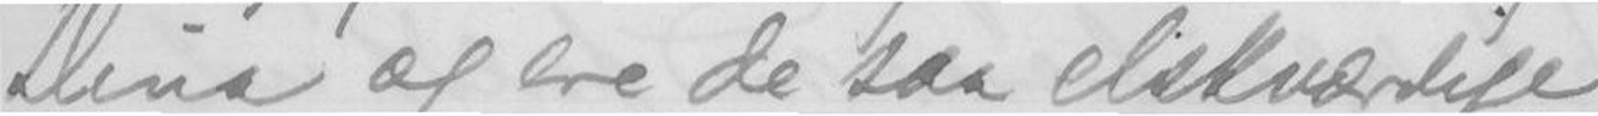Dina og ere de saa elskværdige
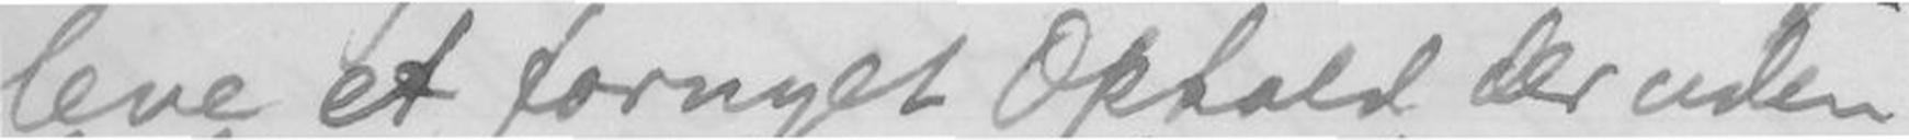leve et fornyet Ophold der uden
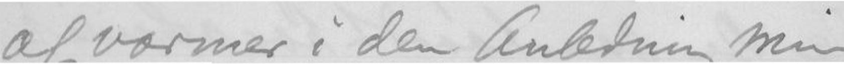og varmer i den Anledning min
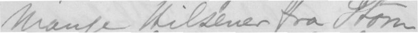Mange Hilsener fra Storm
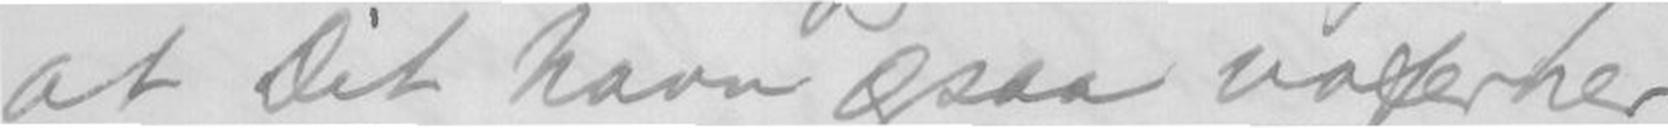at Dit Navn ogsaa voxer her
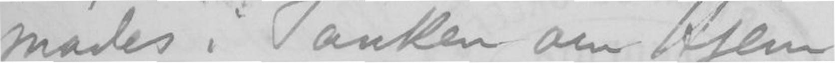mødes i Tanken om Hjem-
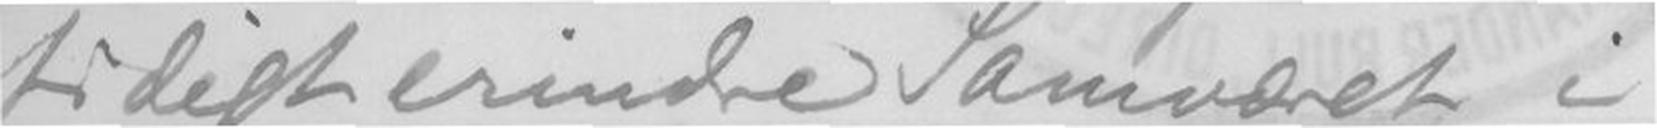tidigt erindre Samværet i
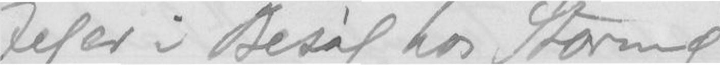Jeg er i Besøg hos Storm og
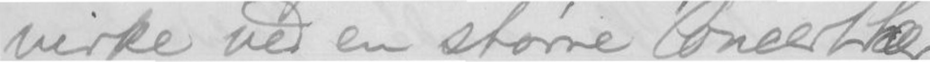virke ved en større Concert her
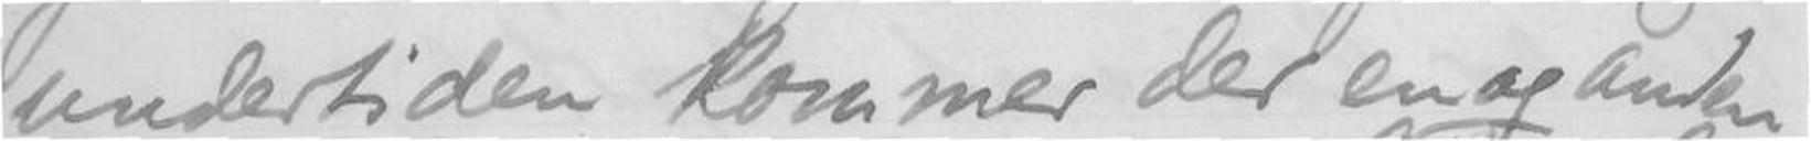undetiden kommer der en og anden
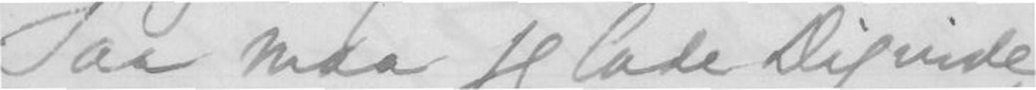Saa maa jeg lade Dig vide
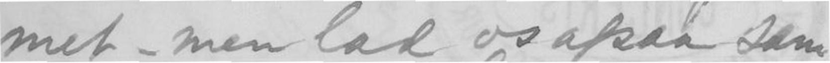met - men lad os ogsaa sam-
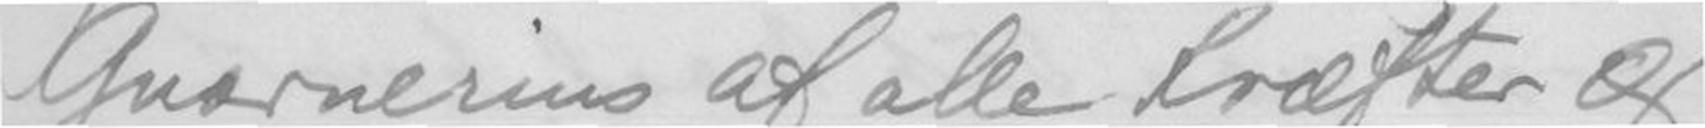Guanerius af alle Kræfter og
